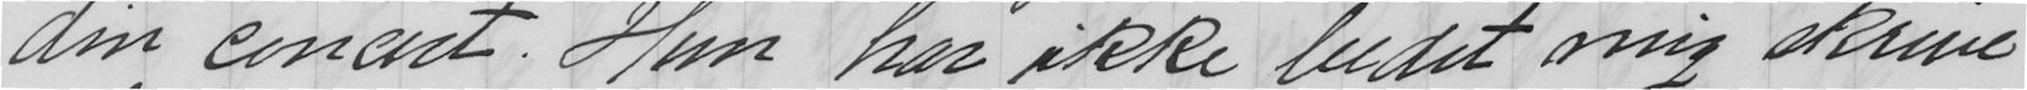din concert. Hun har ikke bedet mig skrive
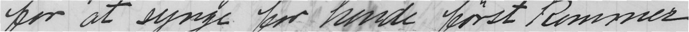for at synge for hende først kommer
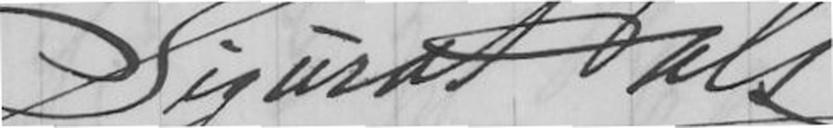Sigurd Hals
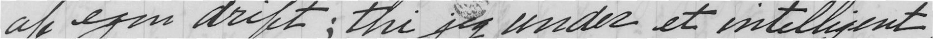af egen drift; thi jeg under et intelligent
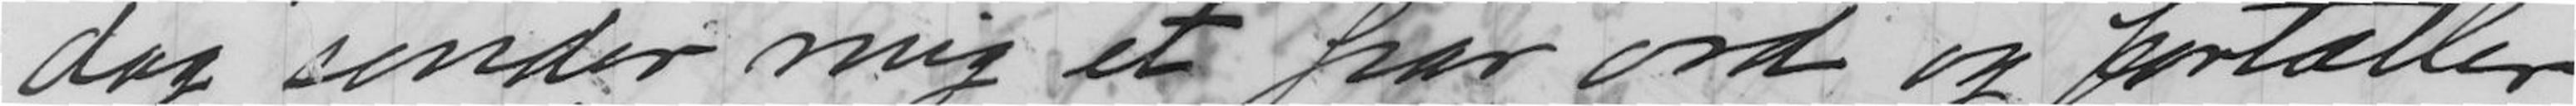dag sender mig et par ord og fortæller,
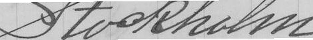Stockholm.
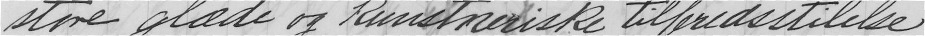store glæde og kunstneriske tilfredsstilelse
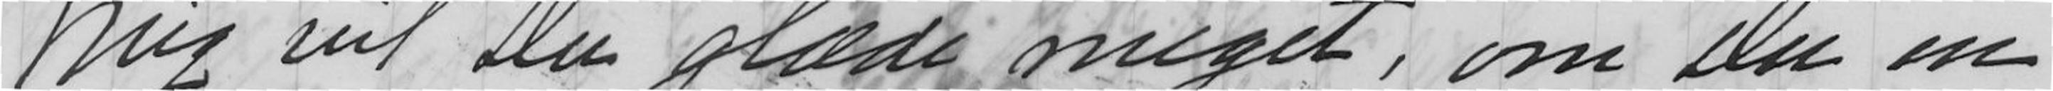Mig vil Du glæde meget, om Du en
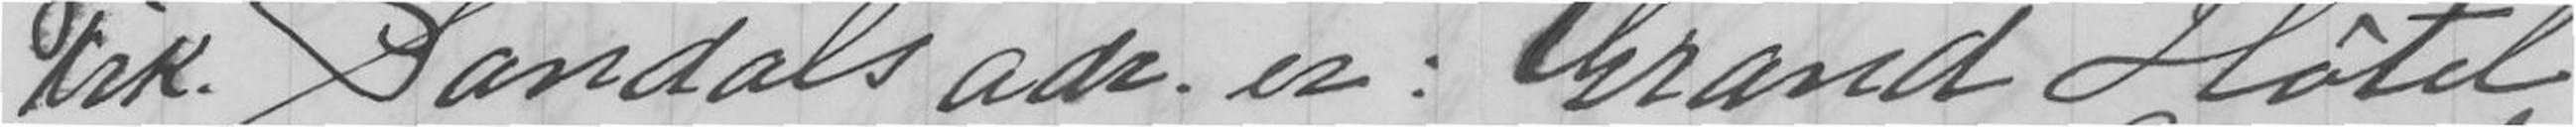Frk. Sandals adr. er: Grand Hôtel
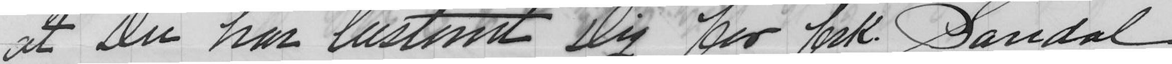at Du har bestemt Dig for frk. Sandal.
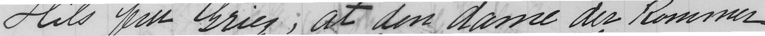Hils fru Grieg; at den dame der kommer
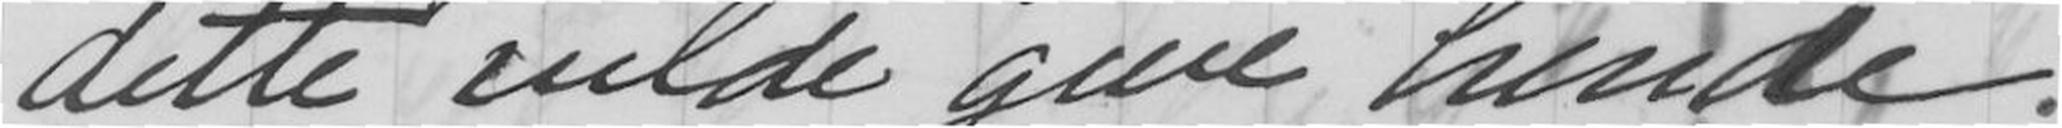dette vilde give hende.
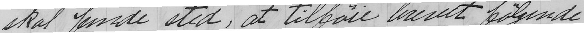skal finde sted, at tilføie brevet følgende
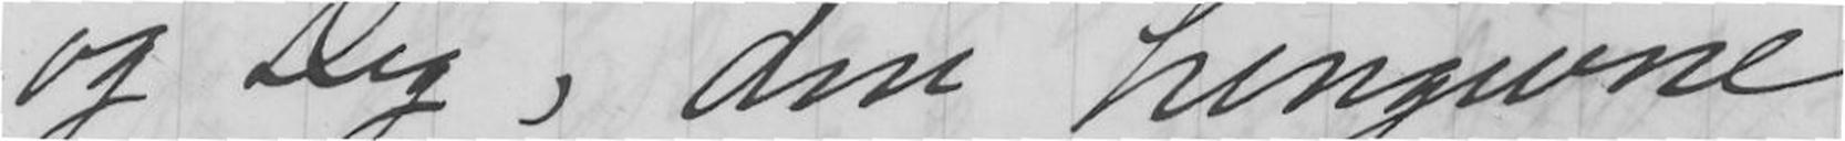og Dig, din hengivne
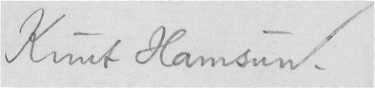Knut Hamsun.
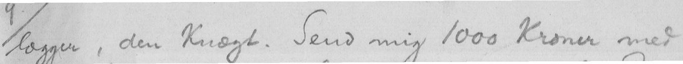lægger, den Knægt. Send mig 1000 Kroner med
